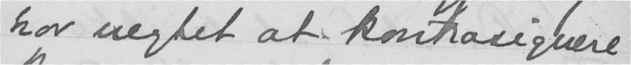har negtet at kontrasignere
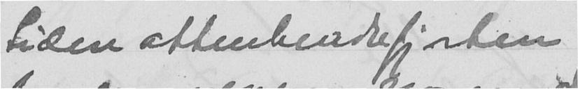Siden attenhundrefjorten
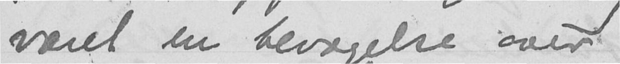været en bevægelse over
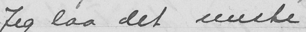Jeg laa det meste
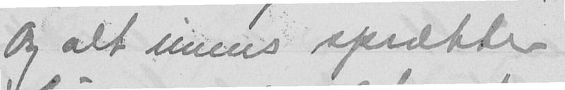Og alt imens sprætter
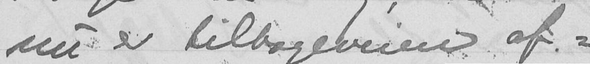nu er tilbageveien af-
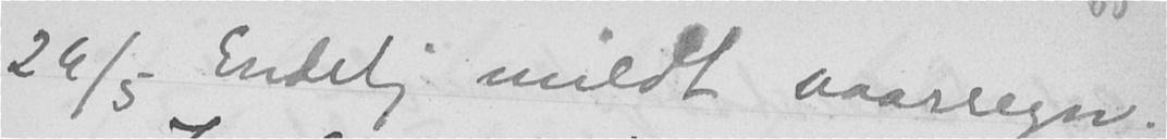26/5 Endelig mildt vaarregn.
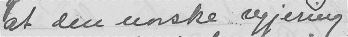at den norske regjering
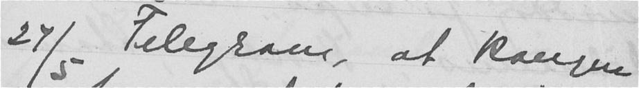27/5 Telegram, at kongen
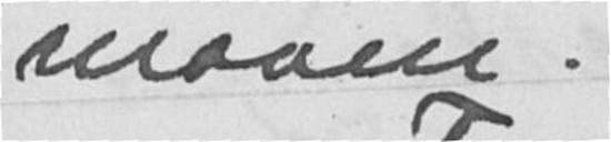maven.
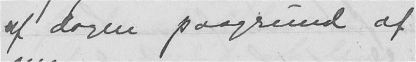af dagen paagrund af
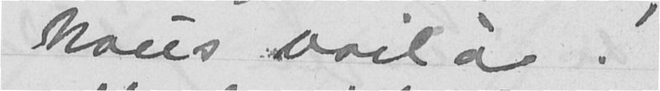Nous voilà!
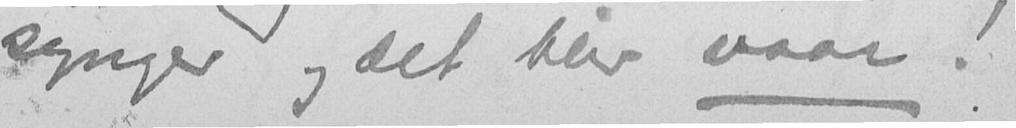synger og det blir vaar!
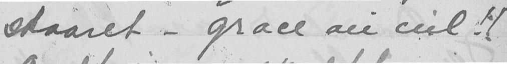skaaret - grace au ciel!!
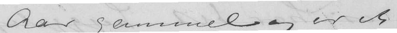Aar gammel og er et
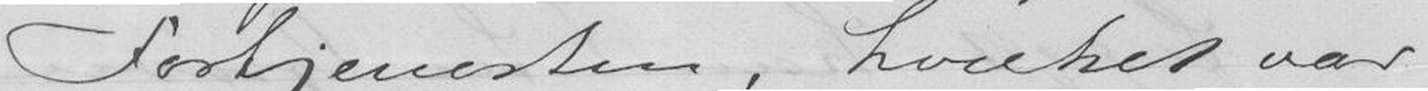Fortjenesten, hvilket var
